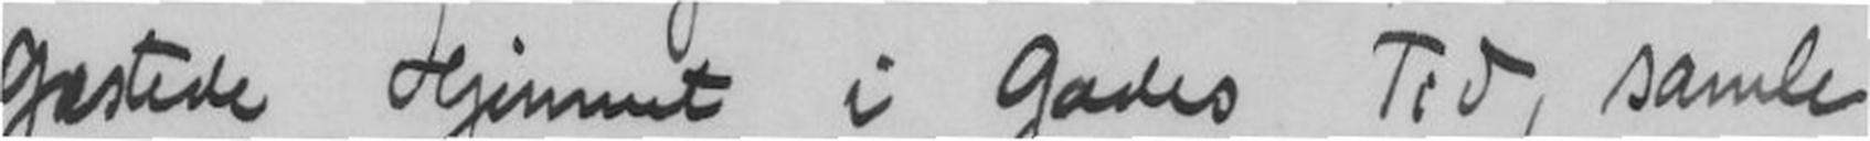Gæstede Hjemmet i Gades Tid, samle
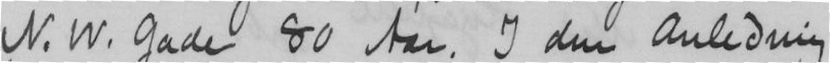N. W. Gade 80 Aar. I den Anledning
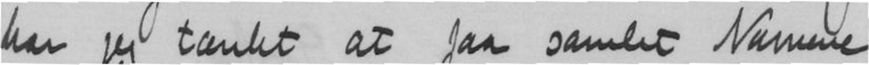har jeg tankt at faa samlet Navnene
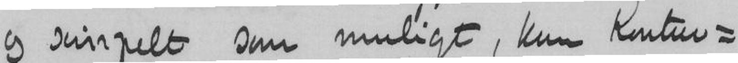og simpelt som muligt, kun kontur-
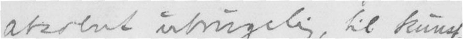absolut ubrugelig, til kunst-
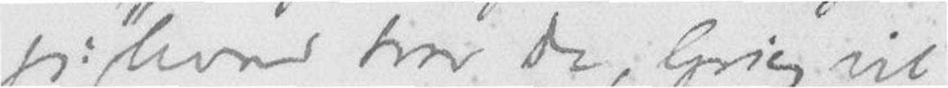jeg: "Hvad tror der, Grieg vil
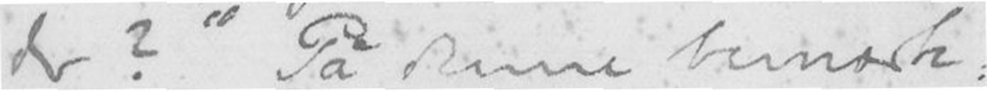der?" På denne bemærk-
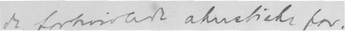der fortvivlende akustiske for-
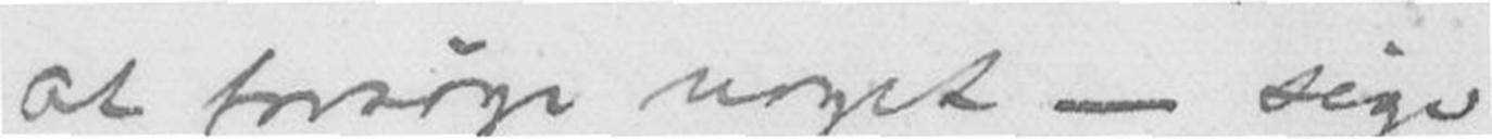at forsøge noget- siger
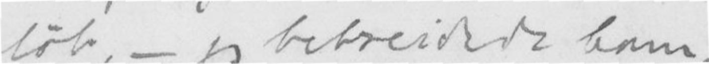løb, jeg bebreidede ham,
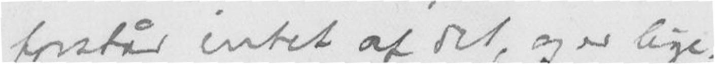forstår intet af det, og er lige-
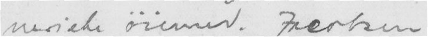neriske øiemed. Jacobsen
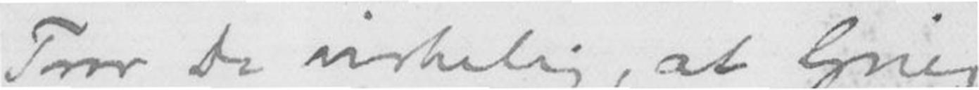Tror Du virkelig, at Grieg
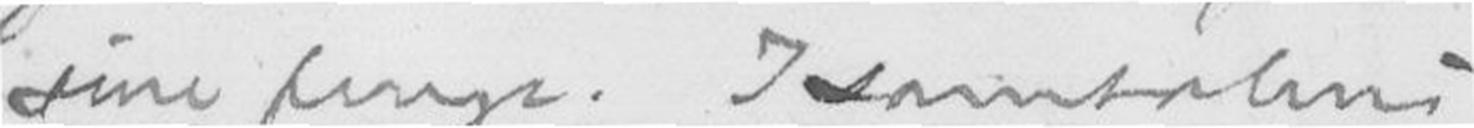sine penger. I samtalens
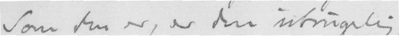Som den er, er den ubrugelig,
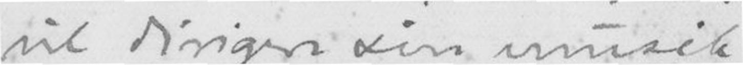vil dirigere sin musik
